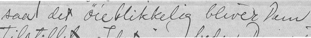saa det øieblikkelig bliver Dem
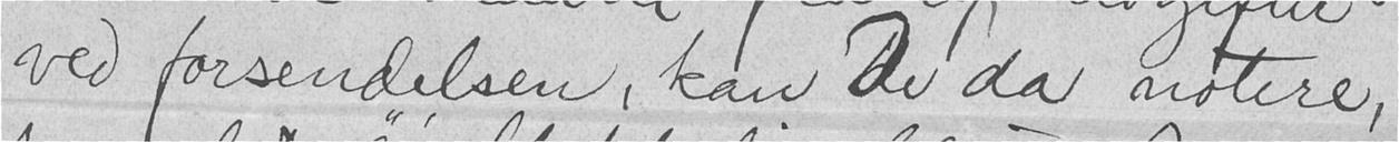ved forsendelsen, kan de da notere,
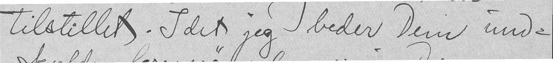tilstillet. I det jeg beder Dem und-
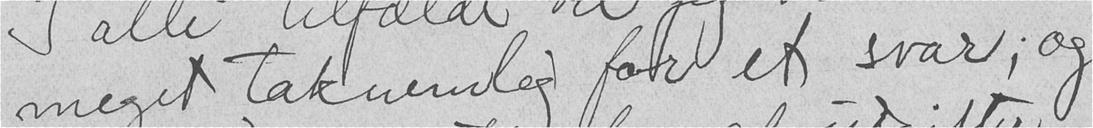meget taknemlig for et svar; og
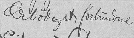Ærbødigst forbundne
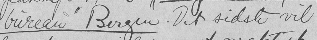bureau" Bergen. Det sidste vil
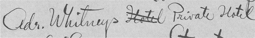Adr. Whitneys  Private Hotel
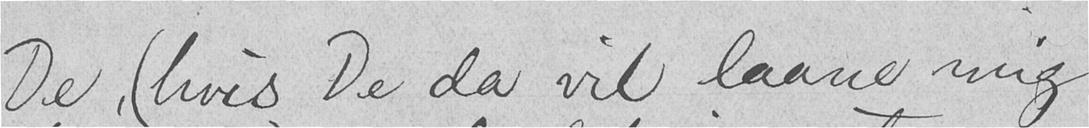De, (hvis De da vil laane mig
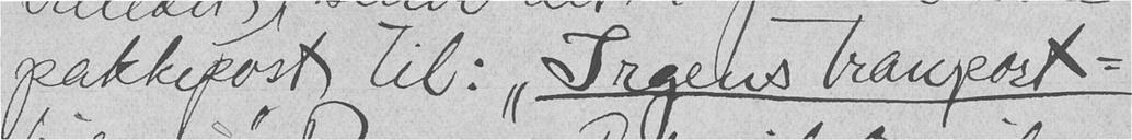pakkepost til: "Irgens tranport-
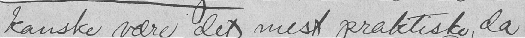kanske være det mest praktiske, da
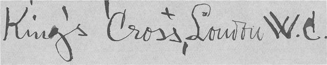King's Cross, London W.C.
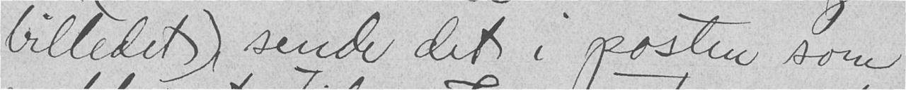billedet), sende det i posten som
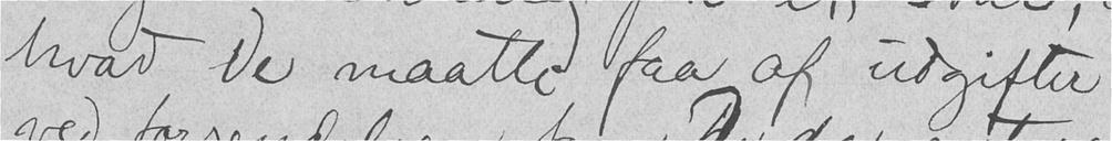hvad De maatte faa af udgifter
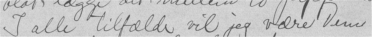I alle tilfælde vil jeg være Dem
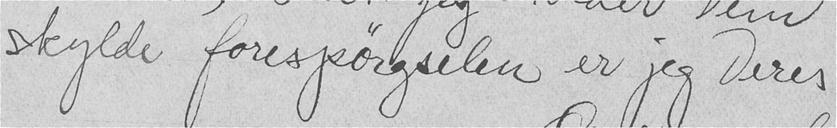skylde forespørgselen er jeg deres
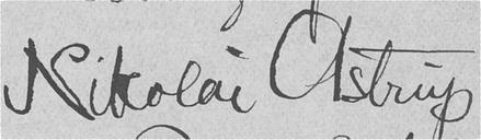Nikolai Astrup
